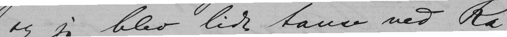og jeg blev lidt tause ved Ra-
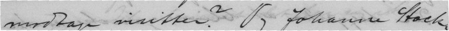modtage visitter? Og Johanne Stock-
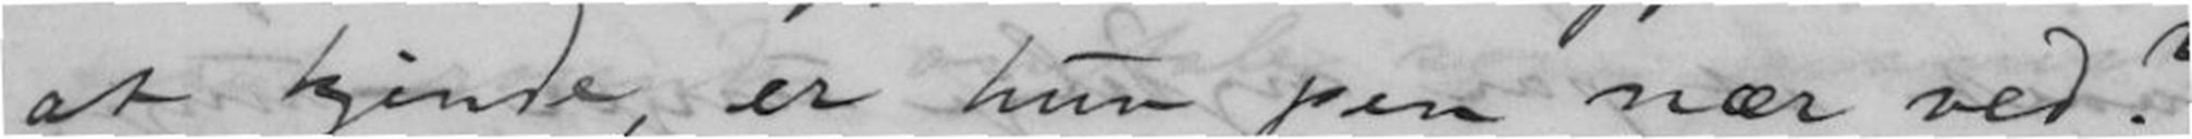at kjende, er hun pen nær ved?
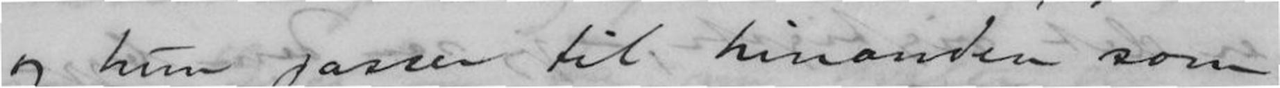og hun passer til hinanden som
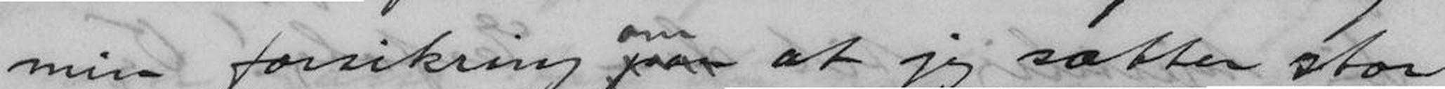min forsikring om paa at jeg sætter stor
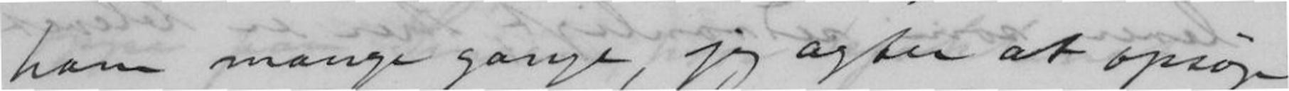ham mange gange, jeg agter at opsøge
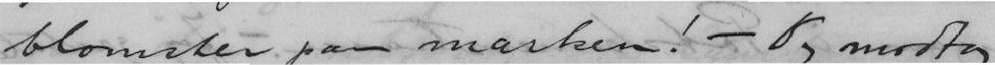blomster paa marken! - Og modtag
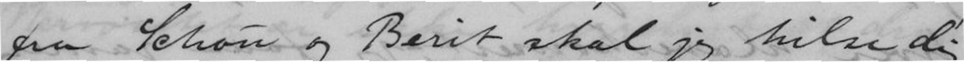fra Schou og Berit skal jeg hilse dig
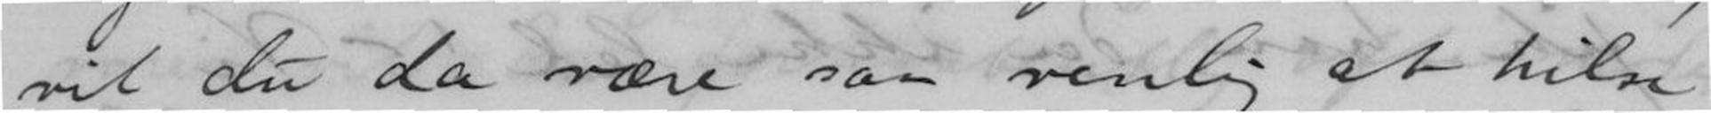vil du da være saa venlig at hilse
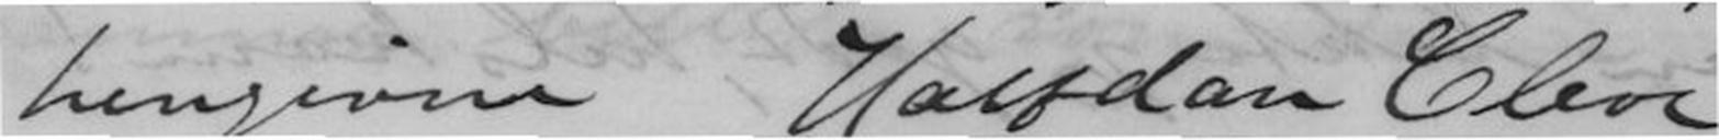hengivne Halfdan Cleve.
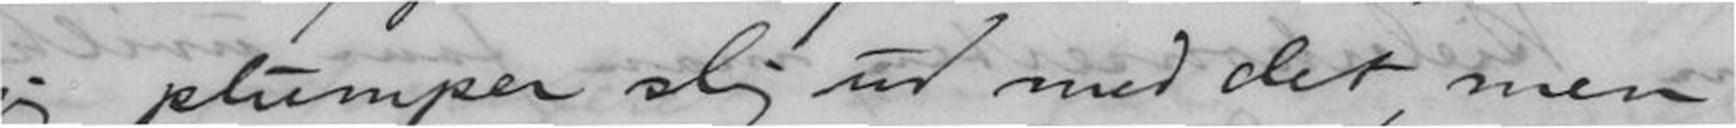jeg plumper slig ud med det, men
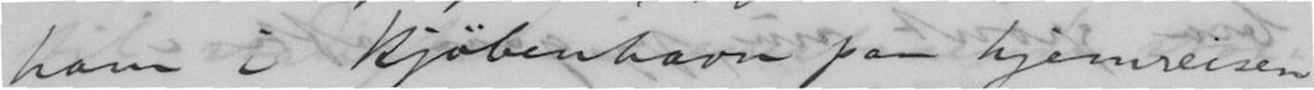ham i Kjøbenhavn paa hjemreisen
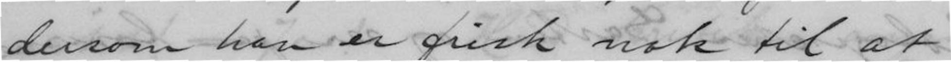dersom han er frisk nok til at
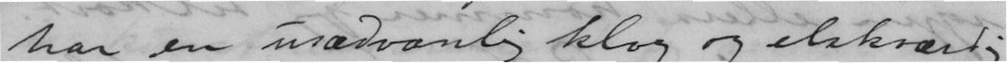har en usædvanlig klog og elskværdig
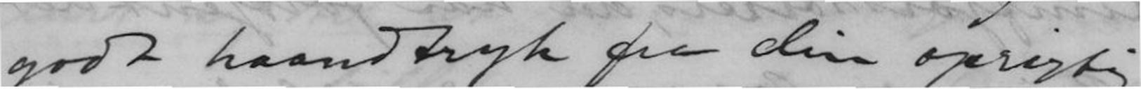godt haandtryk fra din oprigtig
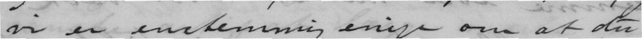vi er enstemmig enige om at du
